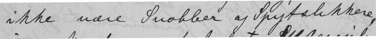ikke være Snobber og Spytslikkere,
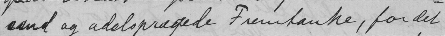aand og adelsprægede Fremtanke, for det
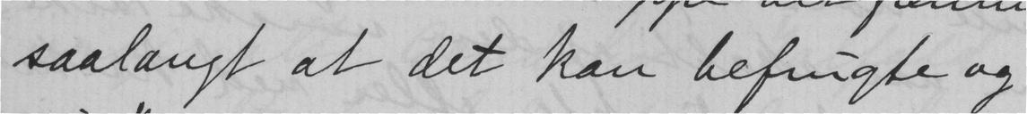saalangt at det kan befrugte og
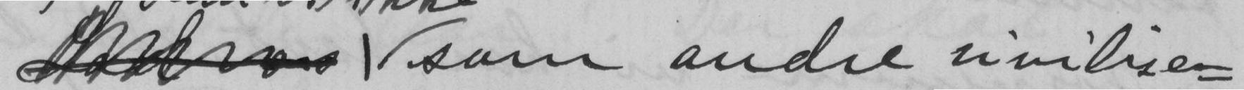som andre ei vilige-
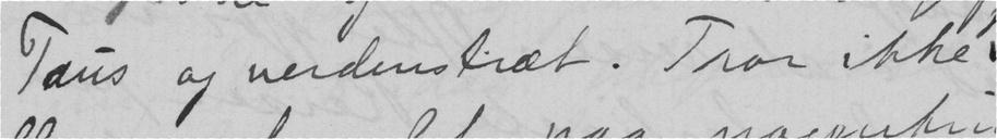Taus og verdenstræt. Tror ikke
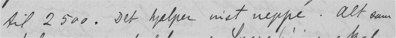til 2 500. Det hjælper vist neppe. Alt som
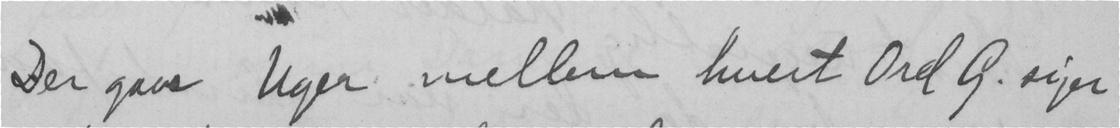Der gaae Uger mellem hvert Ord G. siger
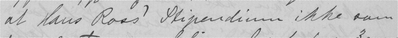at Hans Ross' Stipendium ikke som
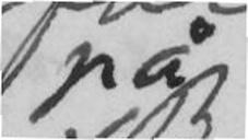på
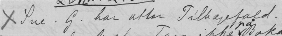x Sne. G. har atter Tilbagefald.
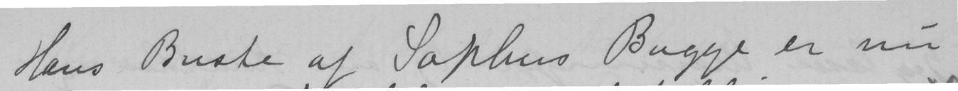Hans Buste af Sophus Bugge er nu
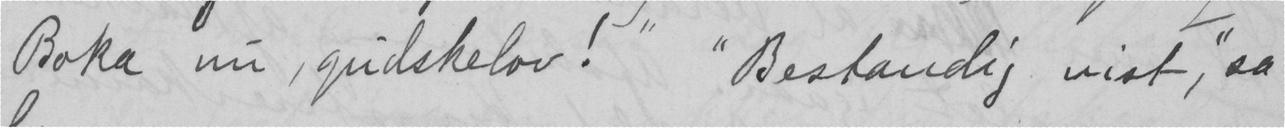Boka nu, gudskelov!" "Bestandig vist," sa
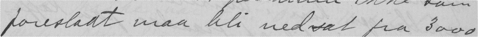foreslaat maa bli nedsat fra 3000
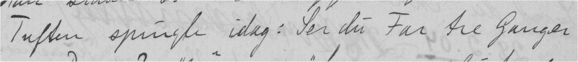Tuften spurgte idag: Ser du Far tre Ganger
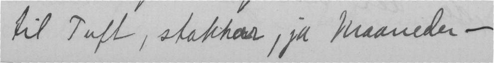til Tuft, stakkar, ja Maaneder -
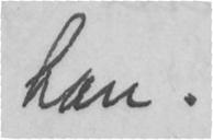han.
